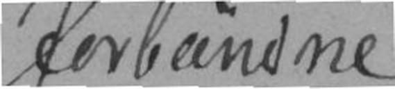Forbøndne
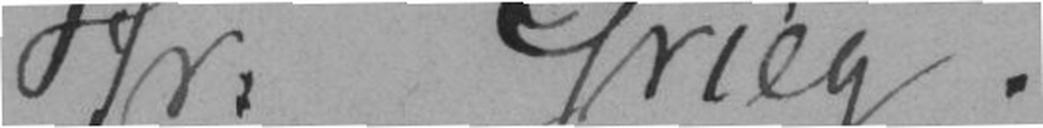Hr. Grieg!
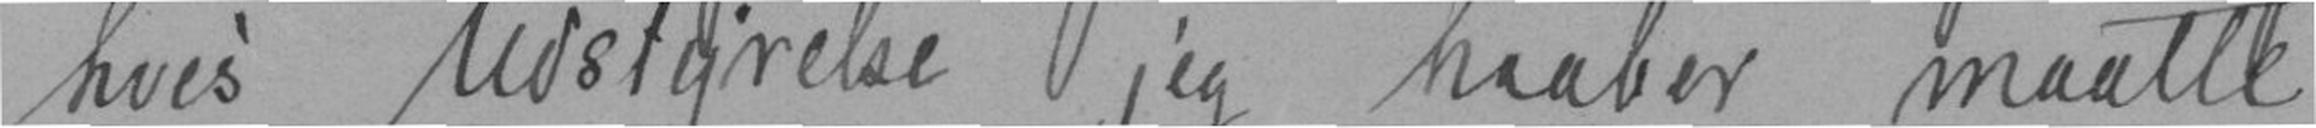hvis udstyrelse jeg haaber maatte
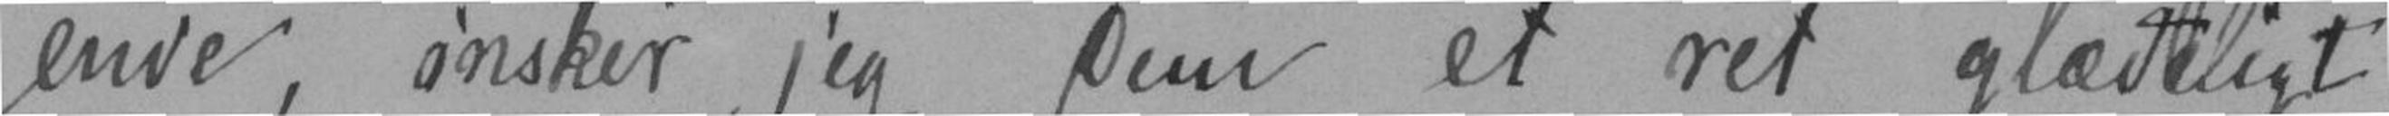ende, ønsker jeg Dem et ret glædeligt
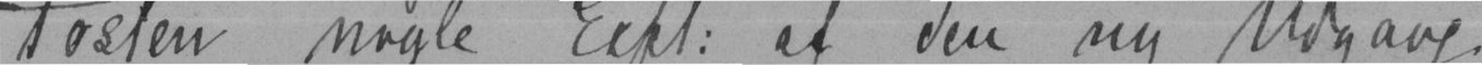Posten nogle Expl: af den ny Mogavl,
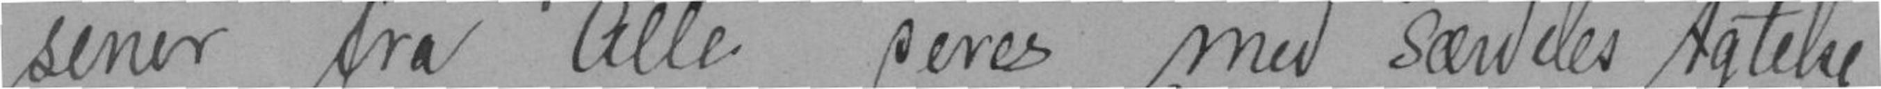sener fra Alle peres med særdeles Agtelse
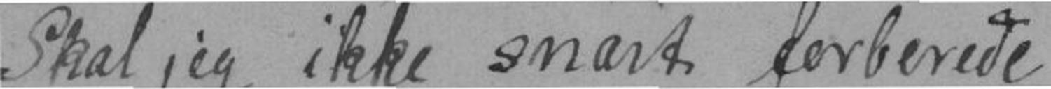Skal jeg ikke snart forberede
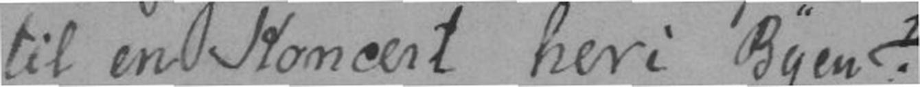til en Koncert heri Byen?
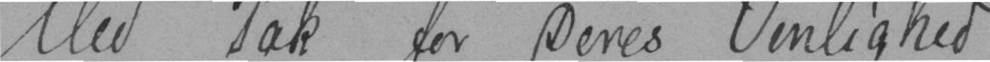Med Tak for Deres Venlighed
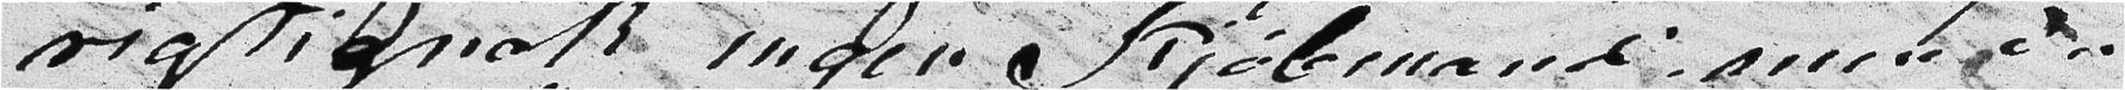rigtignok ingen Kjøbmand, men Du
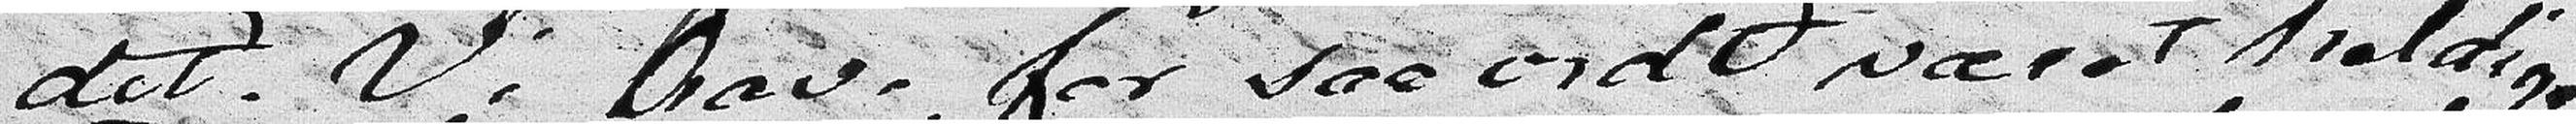det. Vi have for saa vidt været heldige
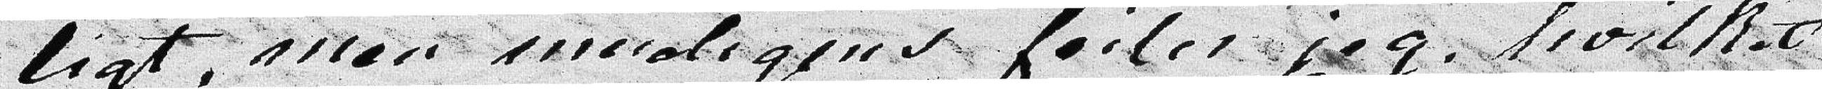ligt, men mueligens feiler jeg, hvilket
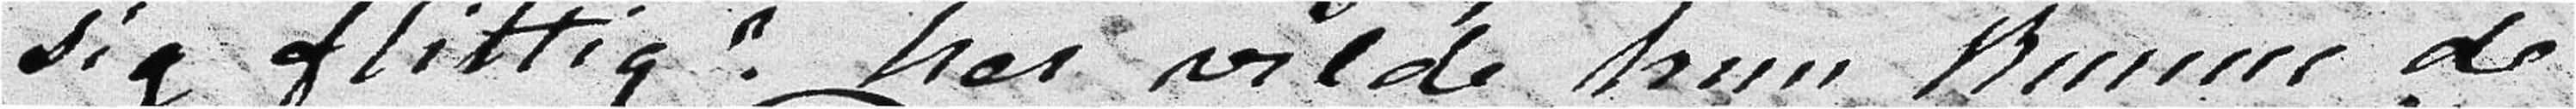sig flittig? her vilde hun kunne de
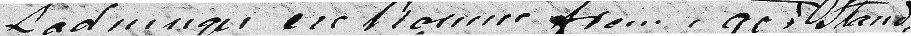Ladninger ere komne frem i god Stand,
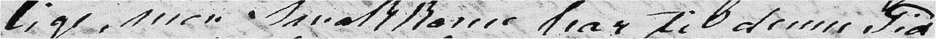lige, men Smakkerne har til denne Tid
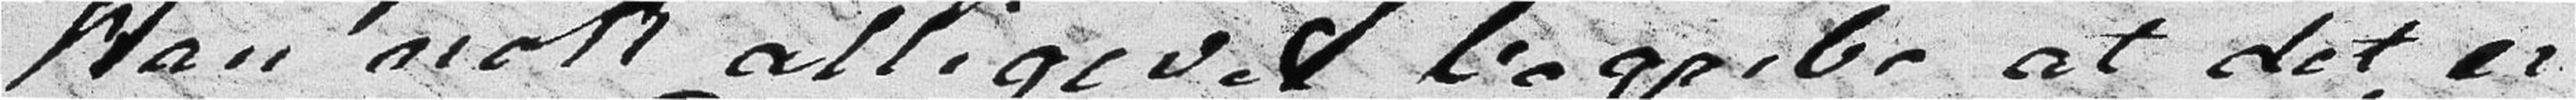kan nok alligevel begribe at det er
